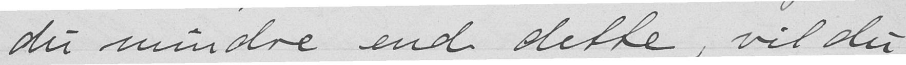du mindre end dette, vil du
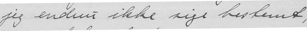jeg endnu ikke sige bestemt;
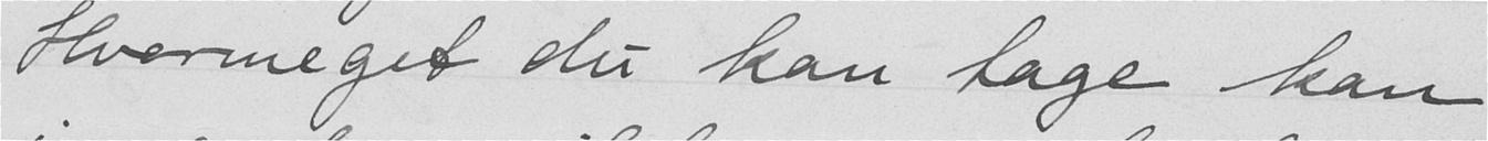Hvormeget du kan tage kan
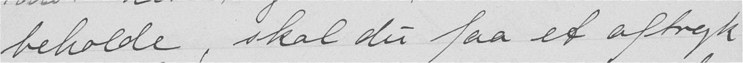beholde, skal du faa et aftryk
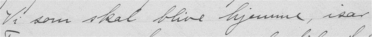Vi som skal blive hjemme, især
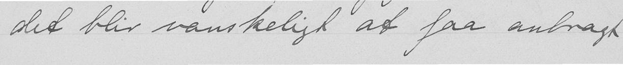det blir vanskeligt at faa anbragt
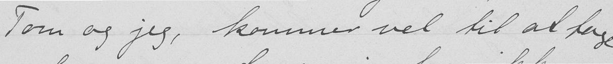Tom og jeg, kommer vel til at tage
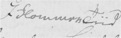I Flommen Tiid
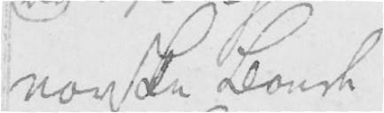norske Bonde
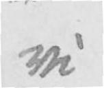vi
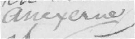Anexerne
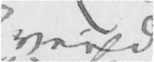vide
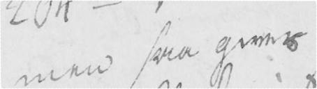men saa gives
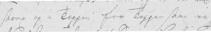strax op i Toppen; fra Toppen saae vi
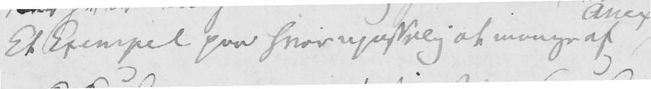Et Exempel paa hvor upasselig at mange af
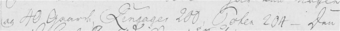og 40 Gaarde, Ringager 200, Toten 204 - Den
